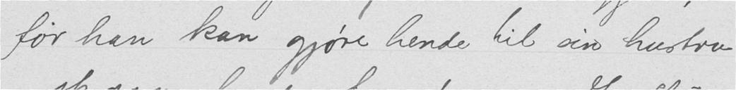før han kan gjøre hende til sin hustru
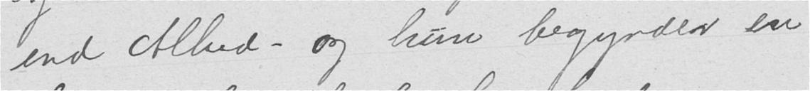end Alhed - og hun begynder en
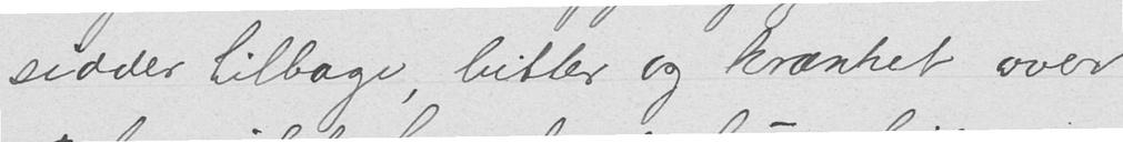sidder tilbage, bitter og krænket over
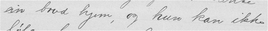en brud hjem, og hun kan ikke
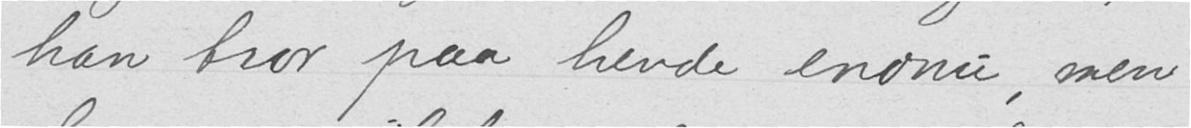han tror paa hende endnu, men
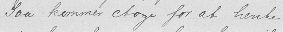Saa kommer Aage for at hente
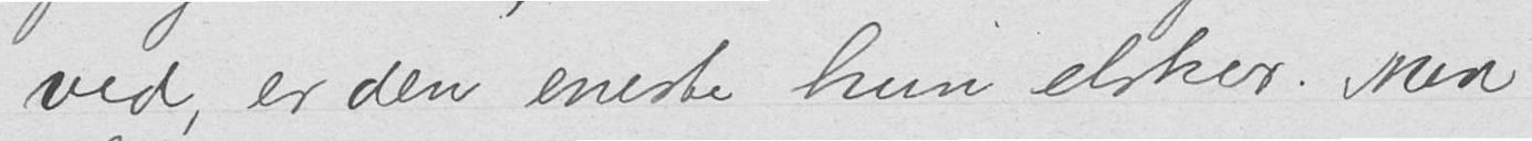ved, er den eneste hun elsker. Men
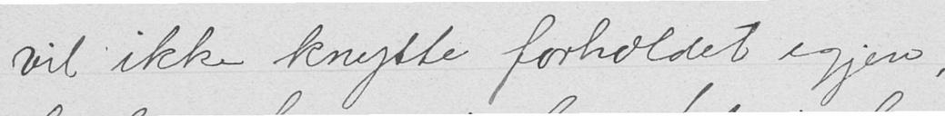vil ikke knytte forholdet igjen,
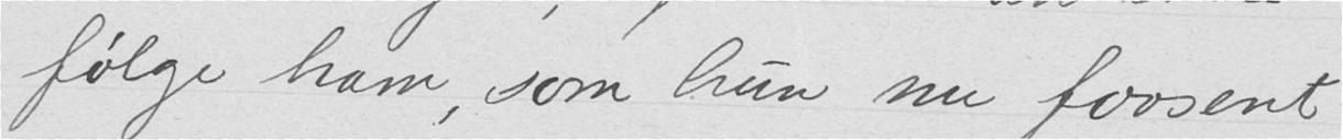følge ham, som hun nu forsent
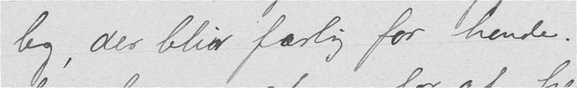leg, der blir farlig for hende.
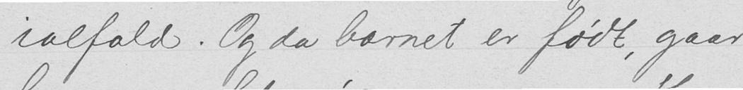ialfald. Og da barnet er født, gaar
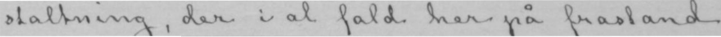staltning, der i al fald her på frastand
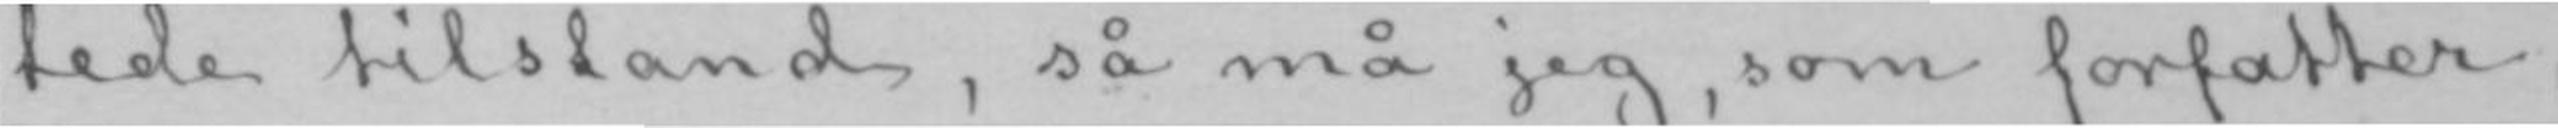tede tilstand, så må jeg, som forfatter,
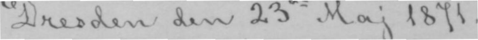Dresden den 23de Maj 1871.
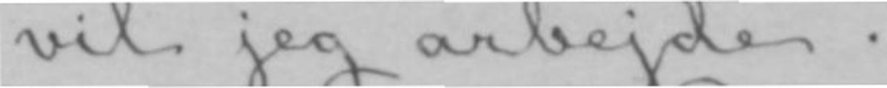vil jeg arbejde.
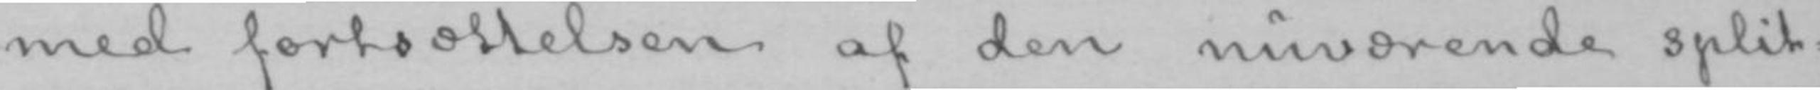med fortsættelsen af den nuværende split-
